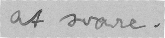at svare.
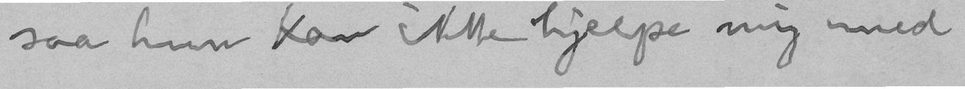saa hun kan ikke hjelpe mig med
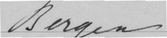Bergen
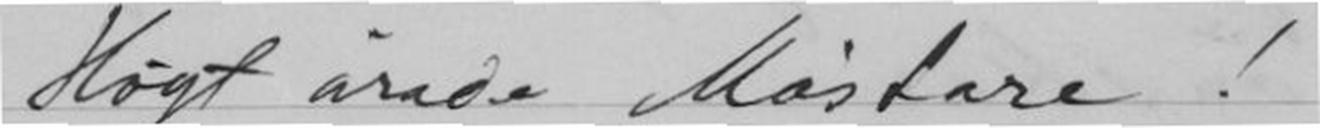Högt ärade Mästare!
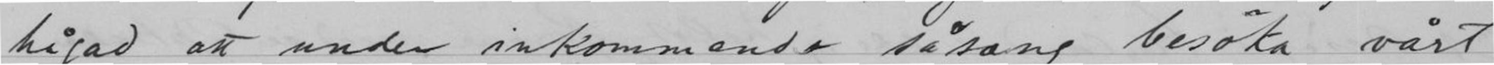hijad att under indkommande säsong besöka vårt
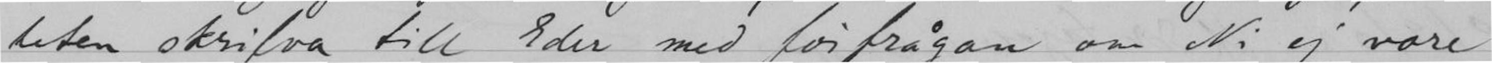heten skrifva till Eder med förfrågan om Ni ej vore
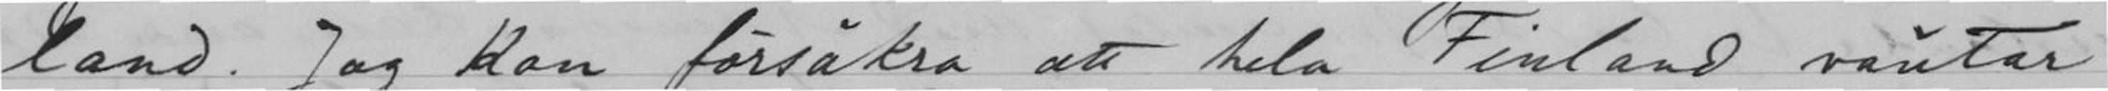land. Jag kan försäkra att hela Finland väntar
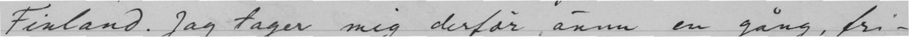Finland. Jag tager mig derför, ännu en gång, fri-
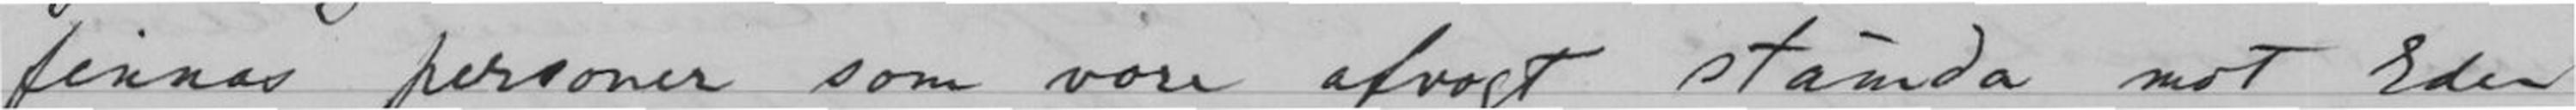finnas personer som vore afvogt stämda mot Eder
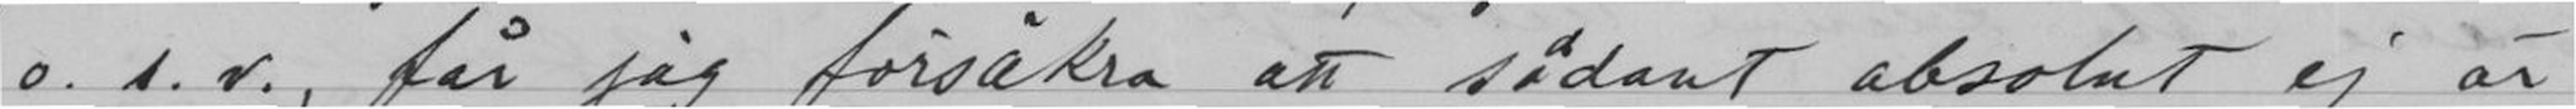o.s.v., får jag försäkra att sådant absolut ej är
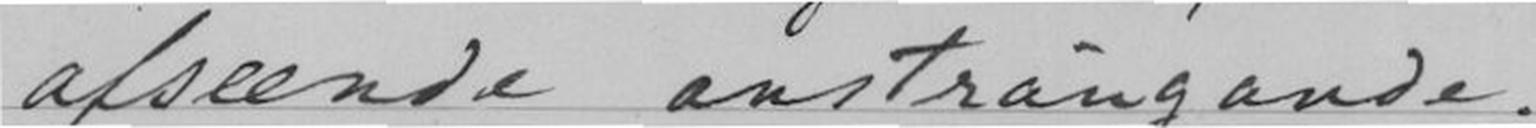afseende ansträngande.
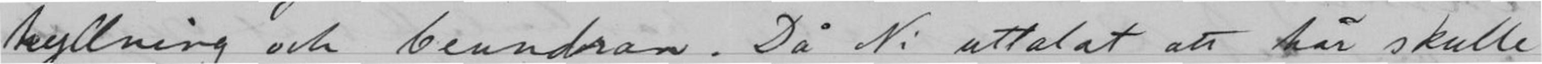hyllning och beundran. Då Ni uttalat att här skulle
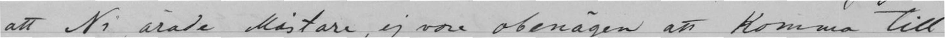att Ni, ärade Mästare, ej vore obenägen att Komma till
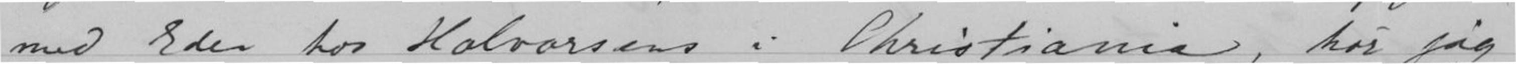med Eder hos Halvorsens i Christiania, hör jag
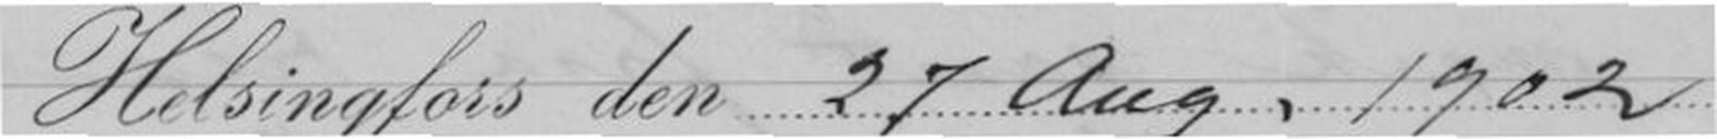Helsingfors den 27 Aug, 1902
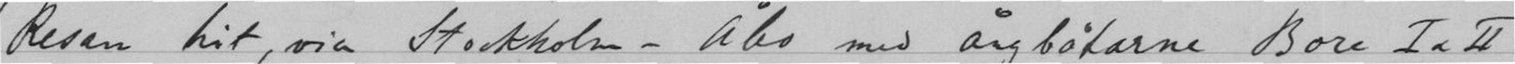Resan hit, via Stockholm - Åbo med ångbåtarne Bore I&II
